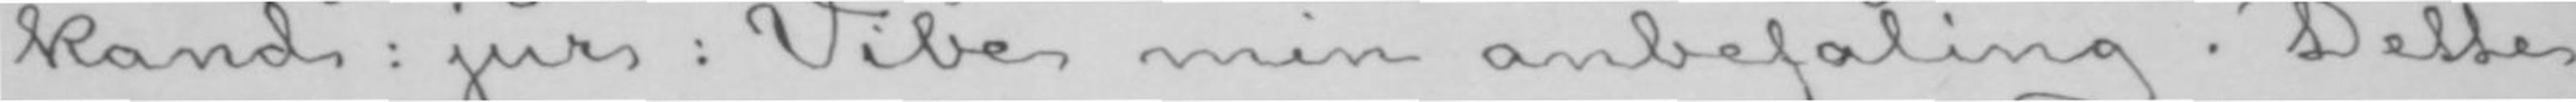kand: jur: Vibe min anbefaling. Dette
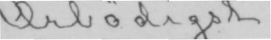Ærbødigst
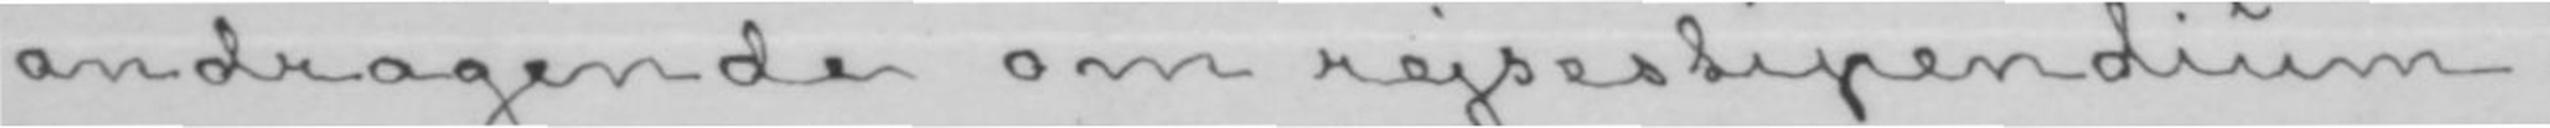andragende om rejsestipendium.
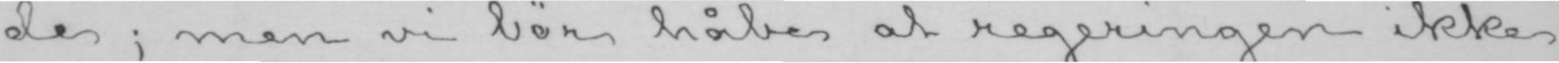de; men vi bør håbe at regeringen ikke
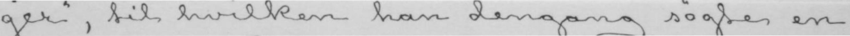ger», til hvilken han dengang søgte en
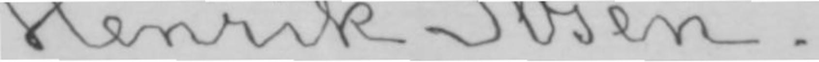Henrik Ibsen.
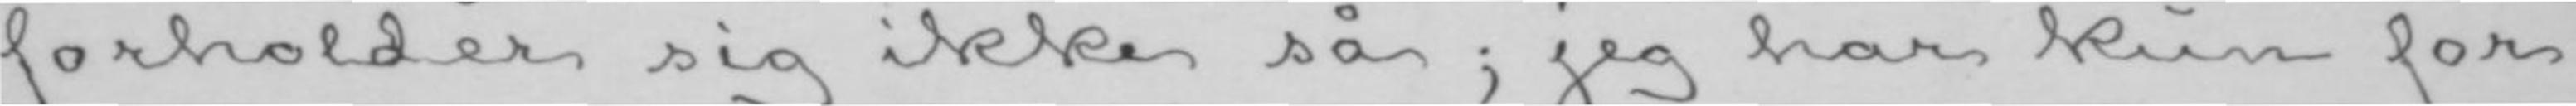forholder sig ikke så; jeg har kun for
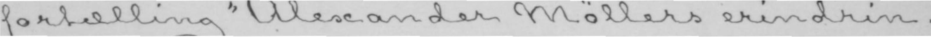fortælling «Alexander Møllers erindrin-
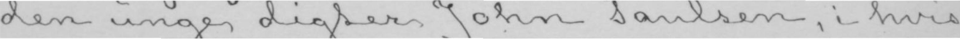den unge digter John Paulsen, i hvis
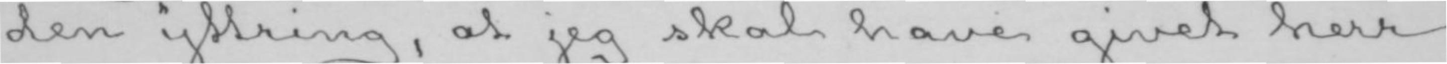den yttring, at jeg skal have givet herr
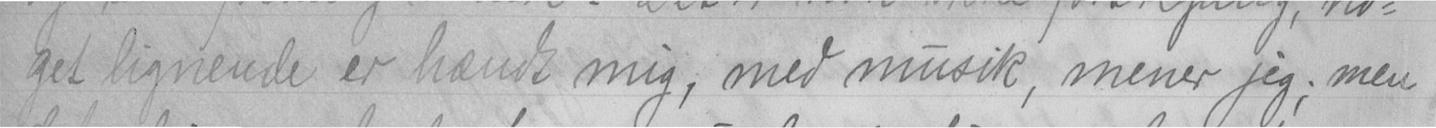get lignende er hændt mig; med musik, mener jeg; men
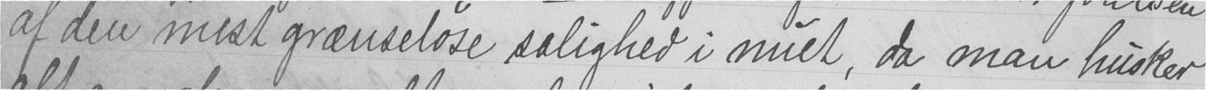af den mest grænseløse salighed i nuet, da man husker
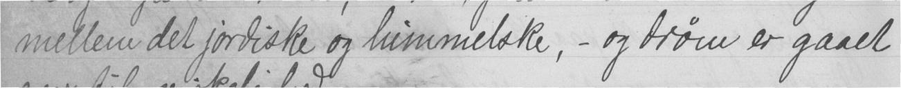mellem det jordiske og himmelske, - og drøm er gaaet
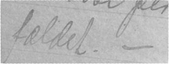fellet. -
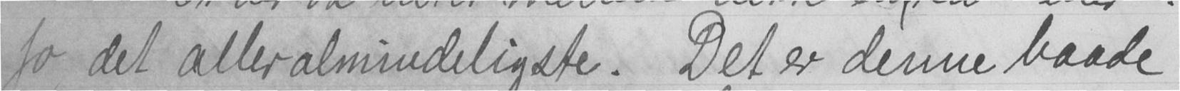Jo, det aller almindeligste. Det er denne baade
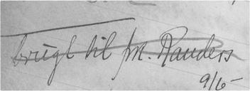brugt til frk. Randers
